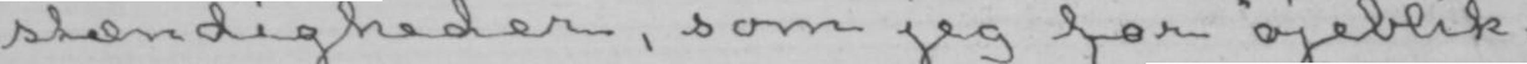stændigheder, som jeg for øjeblik-
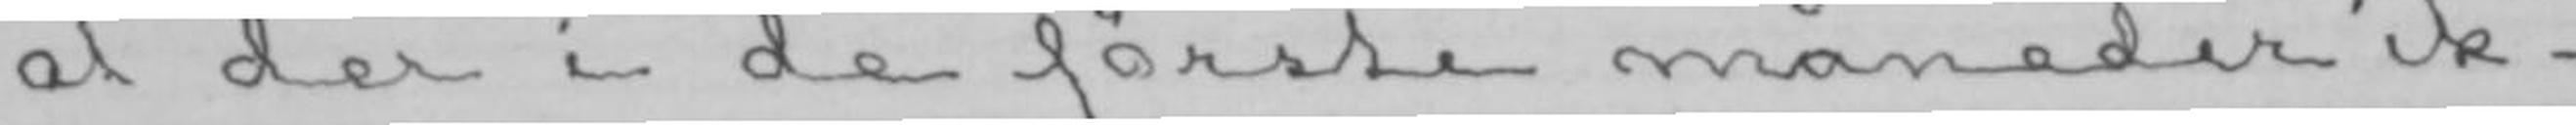at der i de første måneder ik-
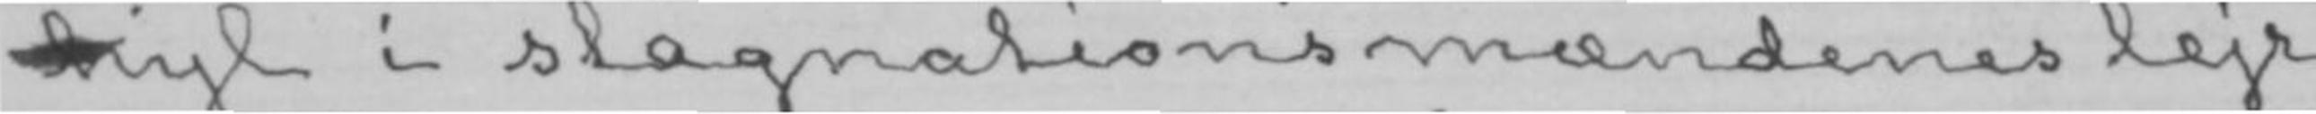hyl i stagnationsmændenes lejr
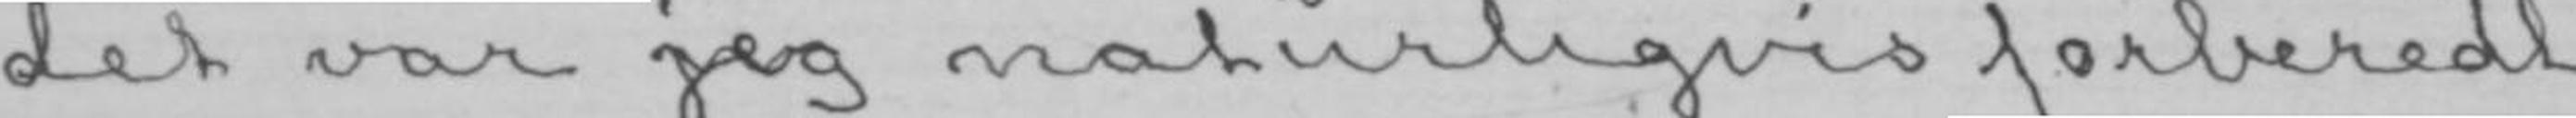det var njeg naturligvis forberedt
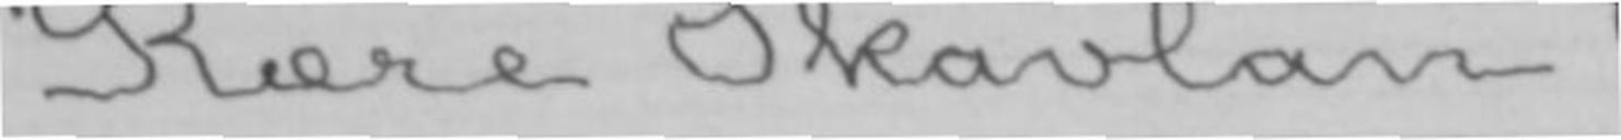Kære Skavlan!
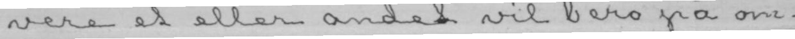vere et eller andet vil bero på om-
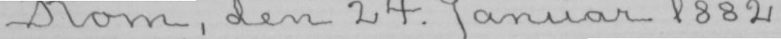Rom, den 24. Januar 1882.
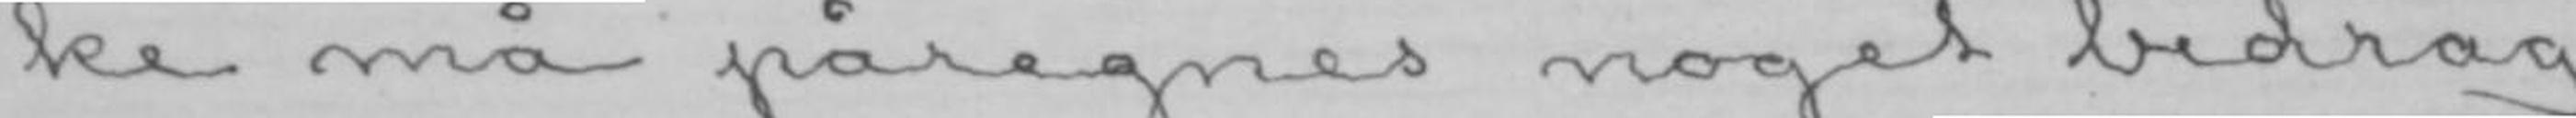ke må påregnes noget bidrag
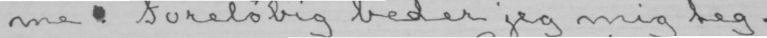me. Foreløbig beder jeg mig teg-
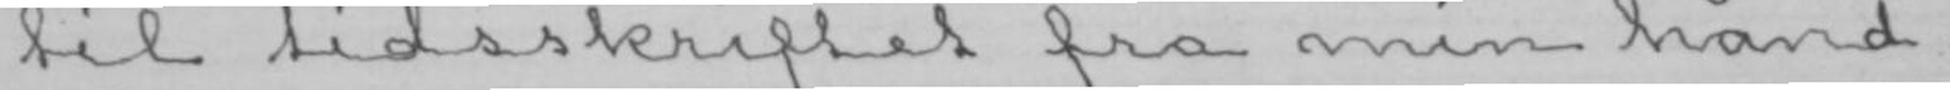til tidsskriftet fra min hånd.
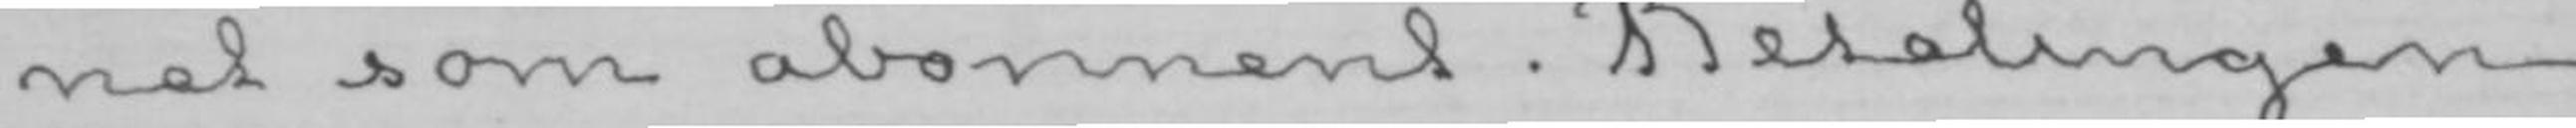net som abonnent. Betalingen
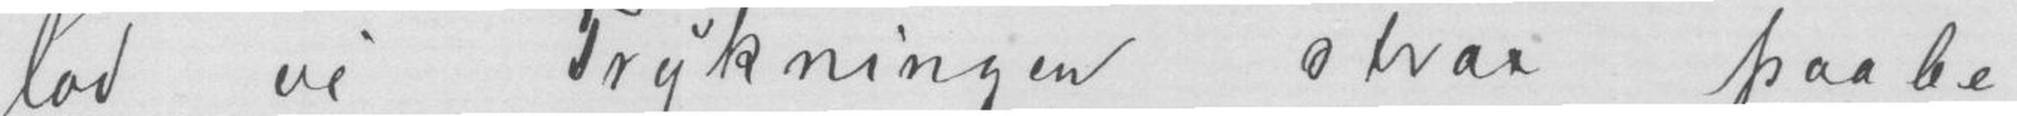lod vi Trykningen strax paabe
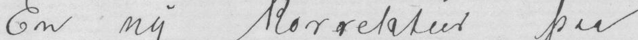En ny Korrektur paa
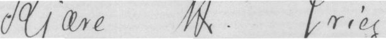Kjære Hr. Grieg!
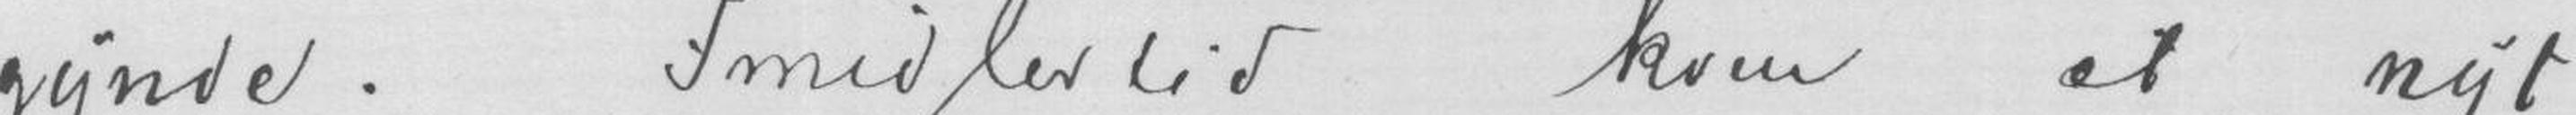gynde. Imidlertid kom et nyt
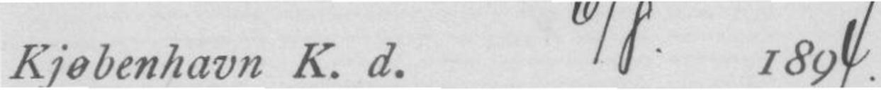Kjøbenhavn K.d. 6/8 1894
